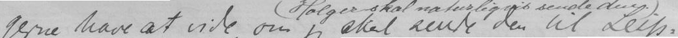gerne have at vide, om jeg skal sende den til Leip-
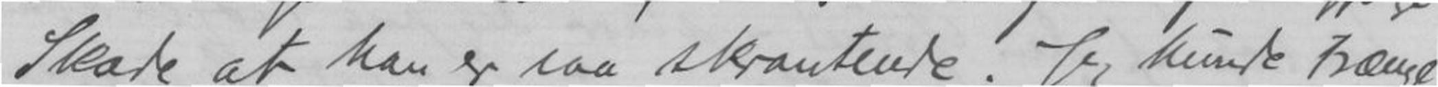Skade at han er saa skrantende. Jeg kunde trænge
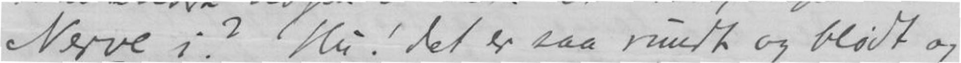Nerve i? Nu! det er saa rundt og
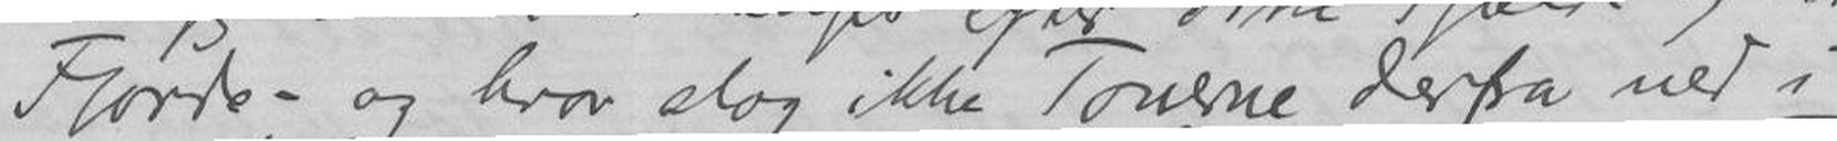Fjorde - og hvor slog ikke Tonerne derfra ned i
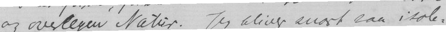og overlegen Natur. Jeg bliver snart saa isole-
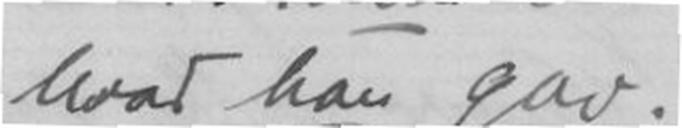hvad han gav!
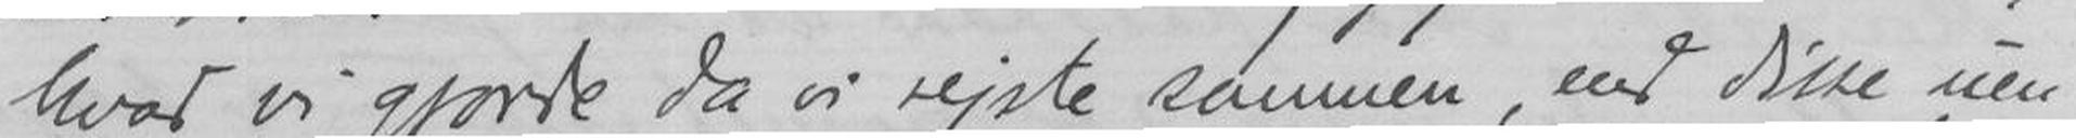hvad vi gjorde da vi rejste sammen, end disse uen-
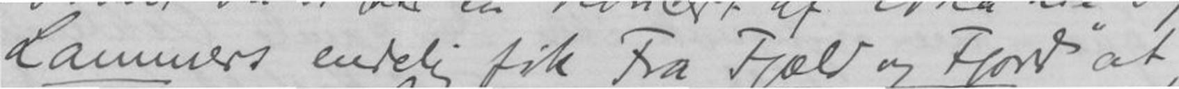Lammers endelig fik Fra Fjæld og Fjord at
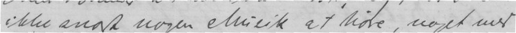ikke snart nogen Musik at høre, noget med
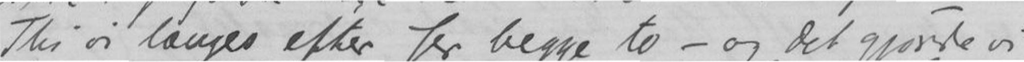Thi vi længes efter Jer begge to - og det gjorde vi
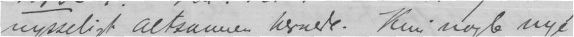blødt og nysseligt altsammen hernede. Kun nogle nye
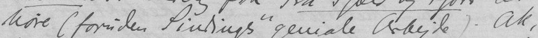høre (foruden Sindings geniale Arbejde). Ak,
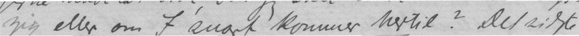zig eller om I snart kommer hertil? Det sidste
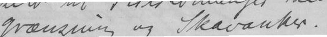grænsing og Skavanker.
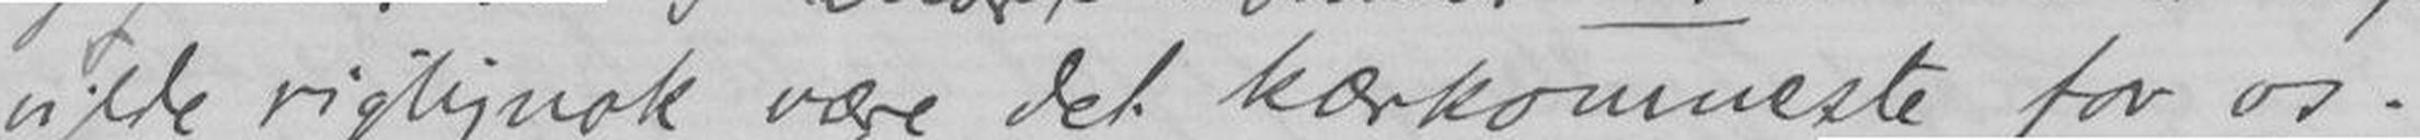vilde rigtignok være det kærkomneste for os.
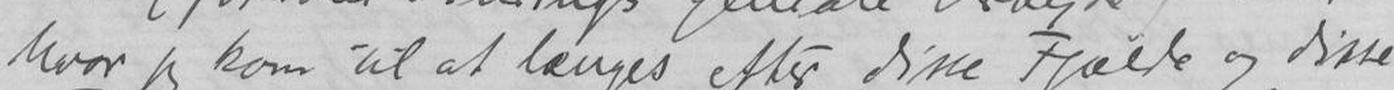hvor jeg kom til at længes efter disse Fjælde og disse
